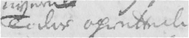Tider oprettede
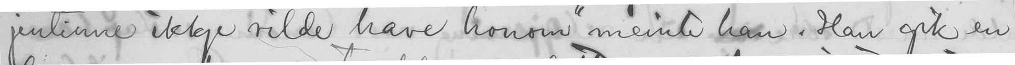jentinne ikkje vilde have honom" meinte han. Han gik en
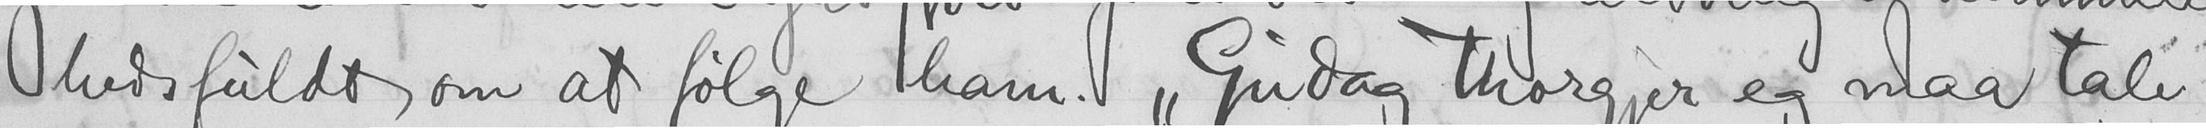hedsfuldt om at følge ham. "Gudag thorgjer eg maa tale
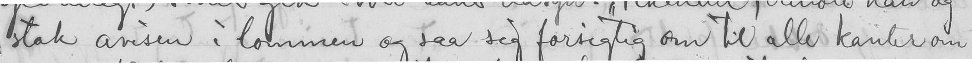stak avisen i lommen og saa sig forsigtig om til aller kanter om
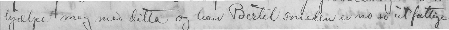hjælpe mig med ditta og han Bertel smeden er no ut fattige
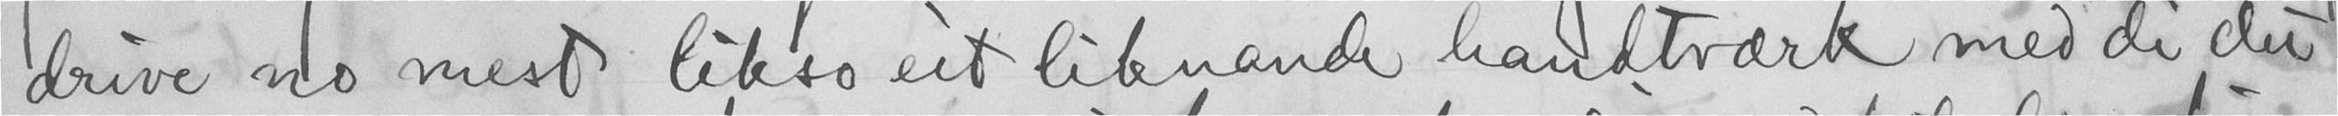drive no mest likso eit liknande handtværk med di du
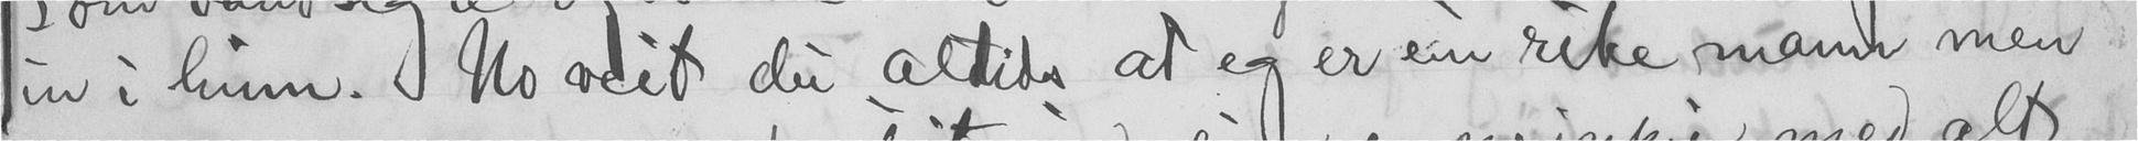in i hum. No veit du altids at eg er en rike mann men
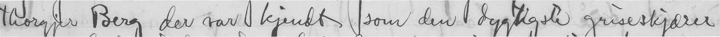thorgjer Berg der var kjendt som den dygtigste griseskjærer
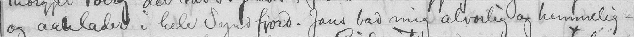og aaklader i hele Syndfjord. Jans bad mig alvorlig og hemmelig-
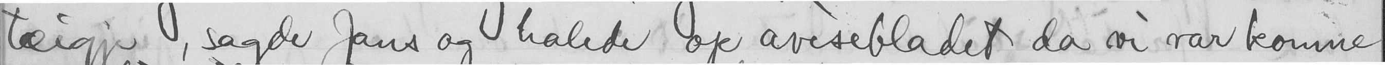tæigje, sagde Jans og halede op avisebladet da vi var komne
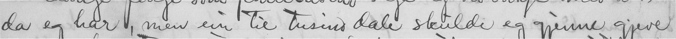da eg har, men ein til tusind dale skulde og gjenne gjeve
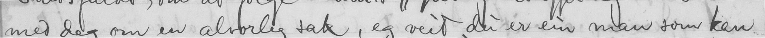med dag om en alvorlig sak, eg veit du er ein man som kan
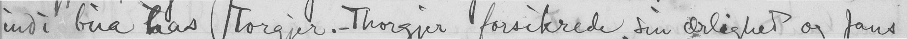ind i bua has torgjer. - thorgjer forsikrede sin ærlighet og Jans
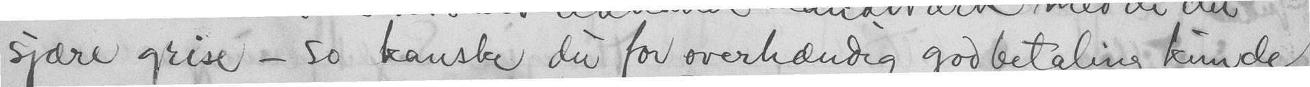sjære grise - so kanske du for overhændig godbetaling kunde
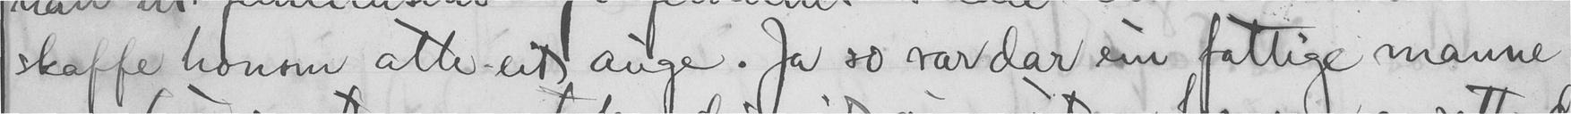skaffe honom atte eit auge. Ja so var dar ein fattige manne
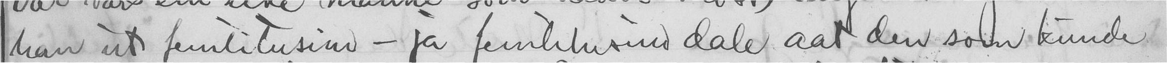han ut femtitusind - ja femtitusind dale aat den som kunde
